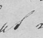at
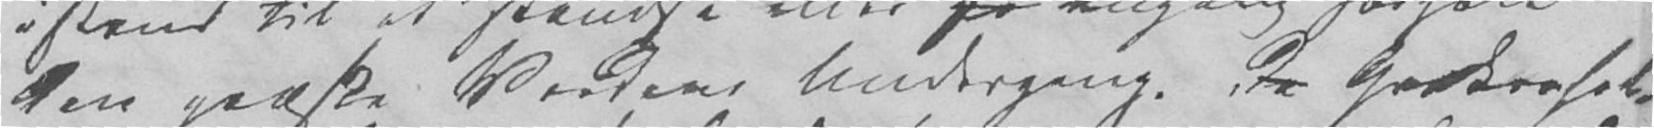den græske Verdens Undergang. De Grækerfol-
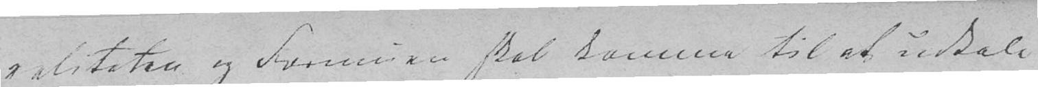raliteten og Formuen skal komme til at udskale
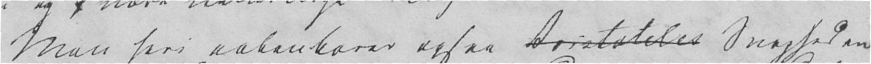Men her aabenbares ogsaa Aristoteles Svagheden
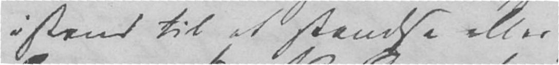istand til at standse eller
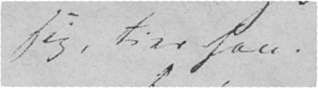sig, tier han.
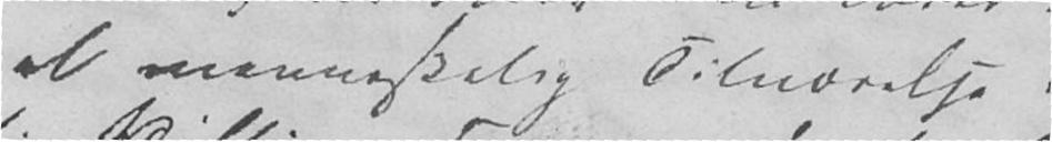al menneskelig Tilværelse
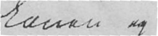Loven og
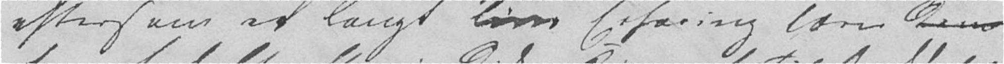eftersom et langt Livs Erfaring lærer dem
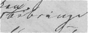bibringe
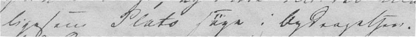ligesom Plato søge i Opdragelsen.
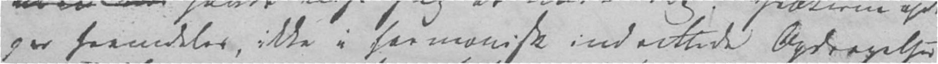ges fremdeles ikke i harmonisk indrettede Opdragelses-
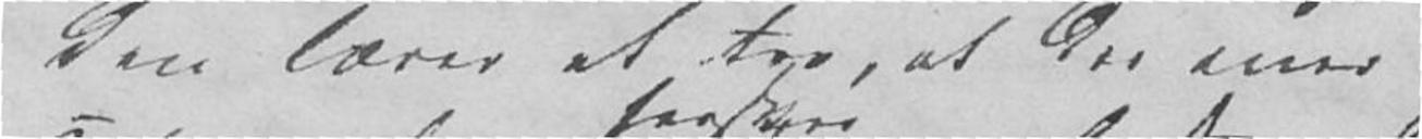den lærer at tro, at der over
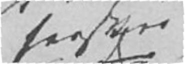hersker
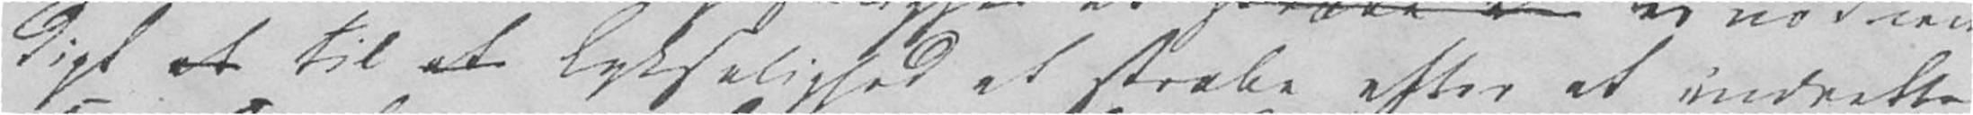digt at til at Lyksalighed at stræbe efter at indrette
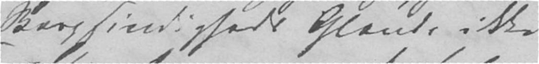Skarpsindigheds Glands ikke
